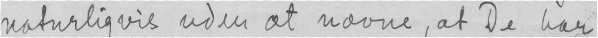naturligvis uden at nævne, at De har
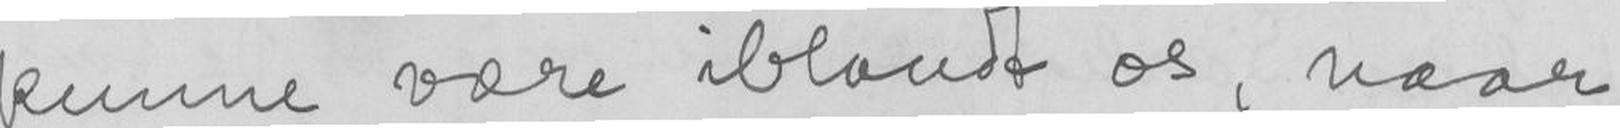kunne være iblandt os, naar
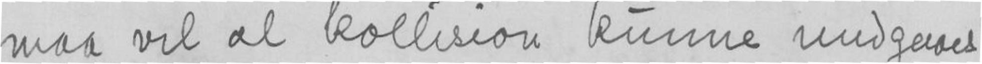maa vel al kollision kunne undgaaes.
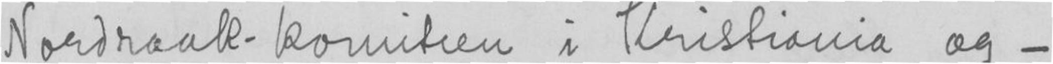Nordraak-komiteen i Kristiania og -
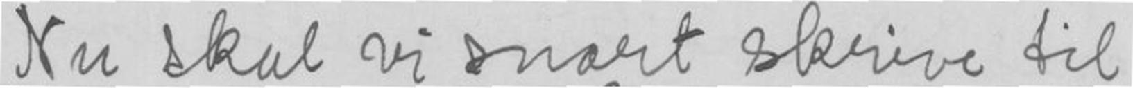Nu skal vi snart skrive til
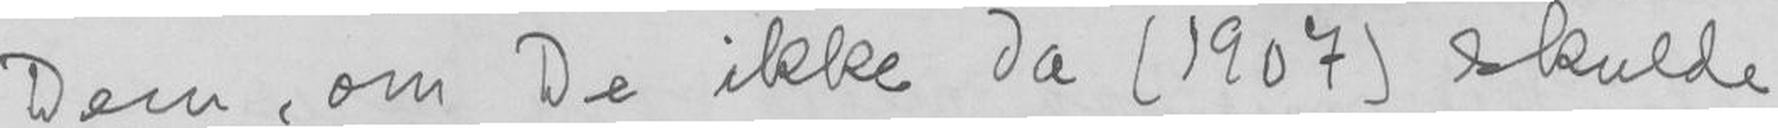Dem, om De ikke da (1907) skulde
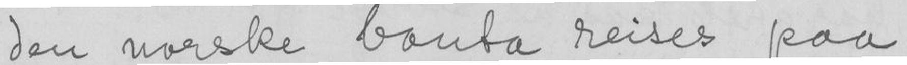den norske bauta reises paa
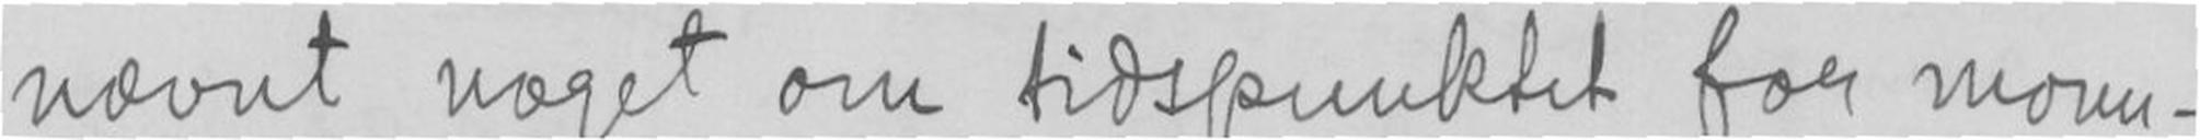nævnt noget om tidspunktet for monu-
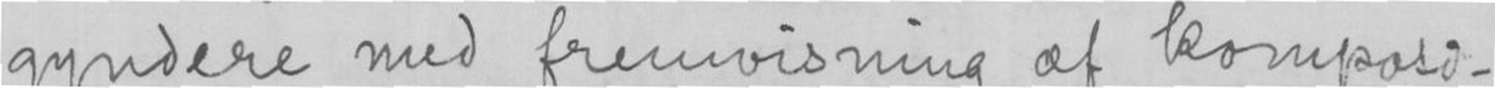gyndere med fremvisning af komposi-
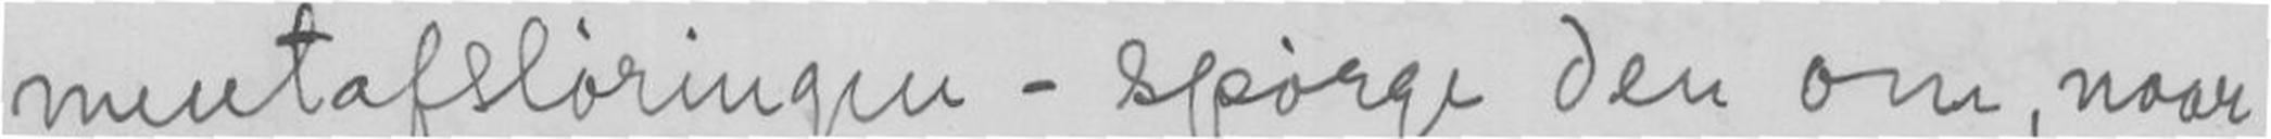mentafsløringen - spørge den om, naar
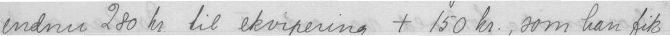endnu 280 kr. til ekvipering + 150 kr., som han fik
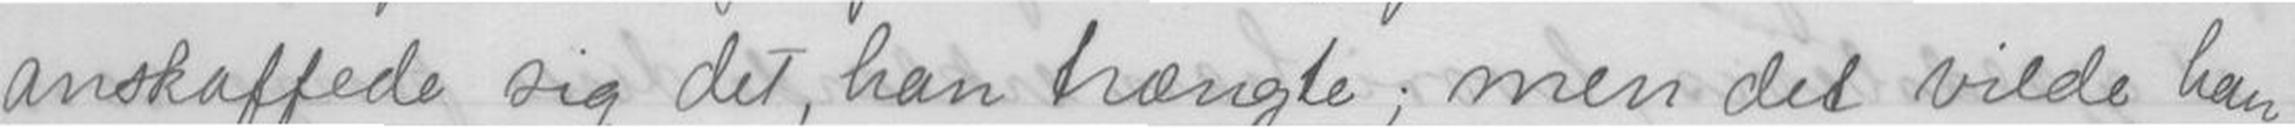anskaffede sig det, han trængte; men det vilde han
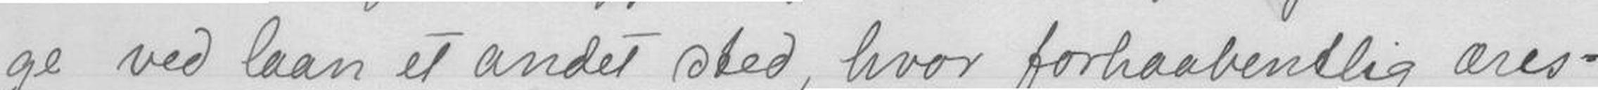ge ved laan et andet sted, hvor forhaabentlig æres-
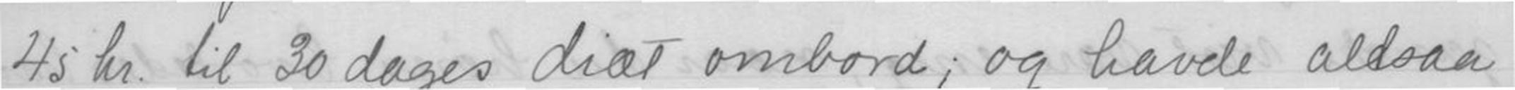45 kr. til 30 dages diæt ombord; og havde altsaa
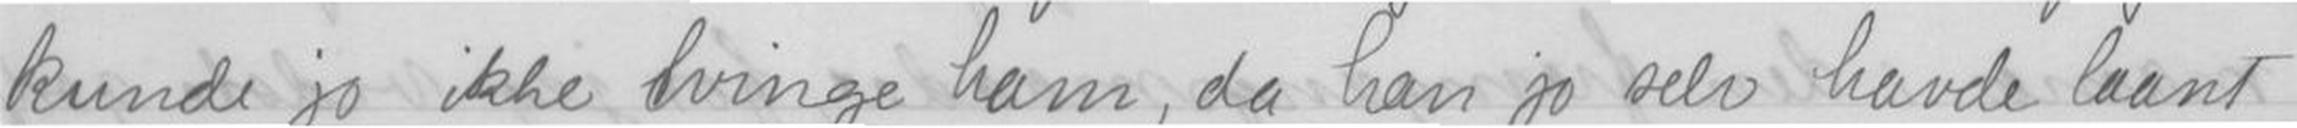kunde jo ikke tvinge ham, da han jo selv havde laant
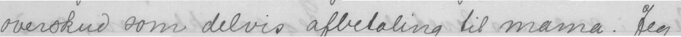overskud som delvis afbetaling til mama. Jeg
